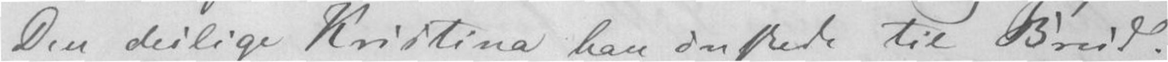Den deilige Kristina han ønskede til Brud.
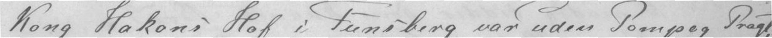Kong Håkons Hof i Tunsberg var uden Pomp og Pragt,
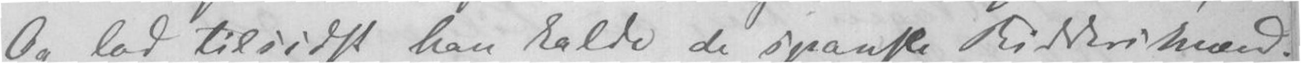Og lod tilsidst han kalde de spanske Riddermænd.
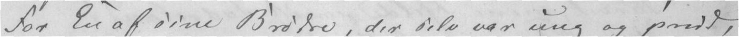For En af sine Brødre, der selv var ung og prud,
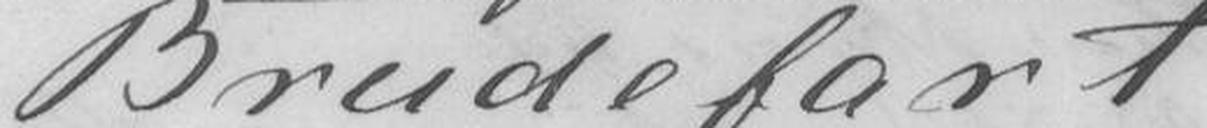Brudefart.
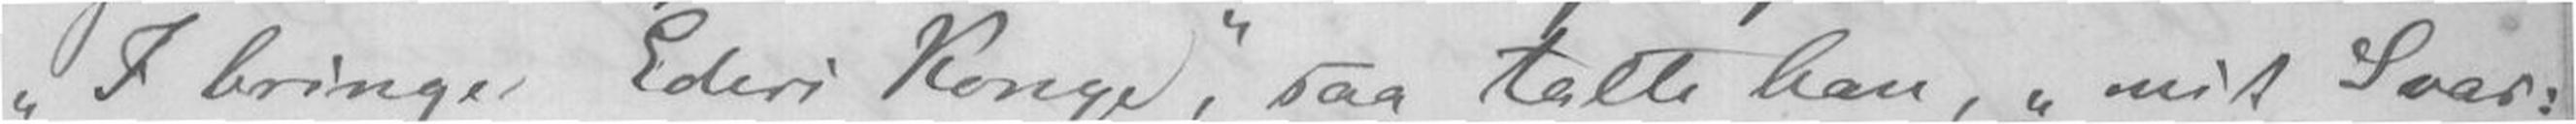I bringer Eders Konge, saa talte han," mit Svar:
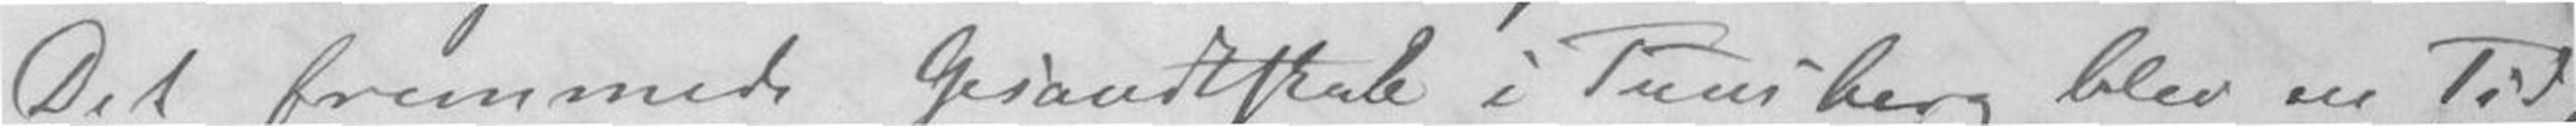Det fremmede Gesandtskab i Tunsberg blev en Tid,
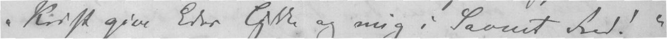Krist give Eder Lykke og mig i Savnet fred!
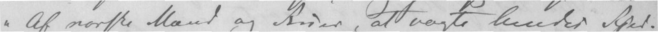"Af norske Mænd og Fruer, at vogte hendes kjed.
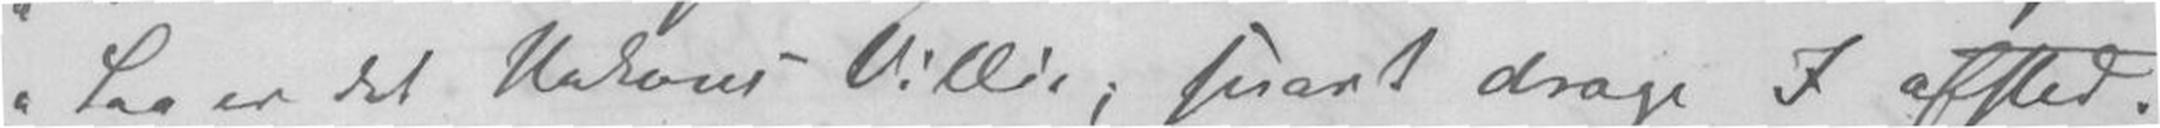"Saa er det Hakons Villie; snart drage I afsted.
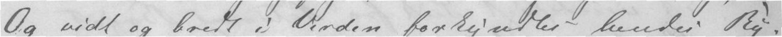Og vidt og bredt i Verden forkyndtes hendes By!
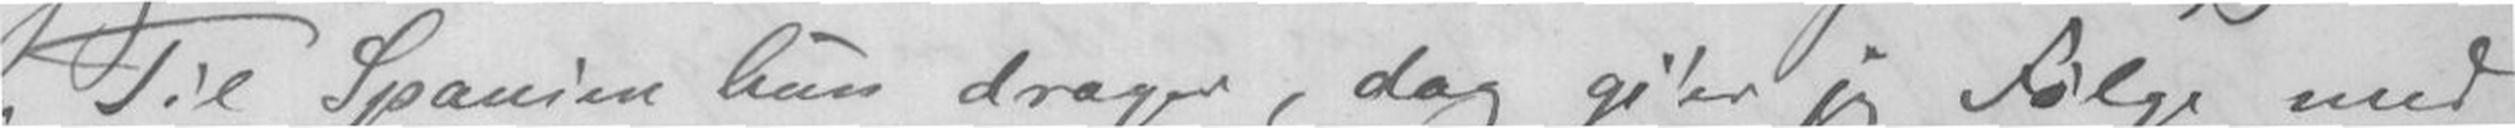Til Spanien hun drage, dog gi'er jeg Følge med
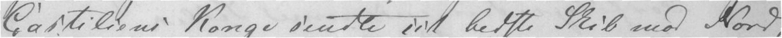Castilisens Konge sendte sit bedste Skib mod Nord,
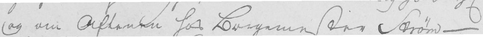og om Aftenen hos Borgemester Strøm -
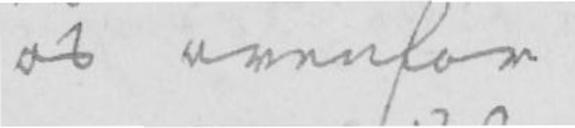os ovenfor
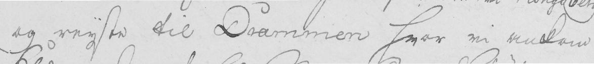og reyste til Drammen hvor vi ankom
